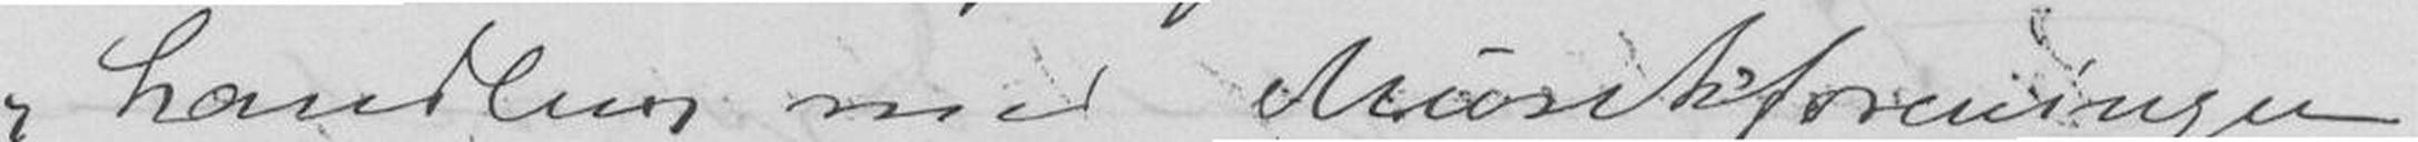handling med Musikforeningen
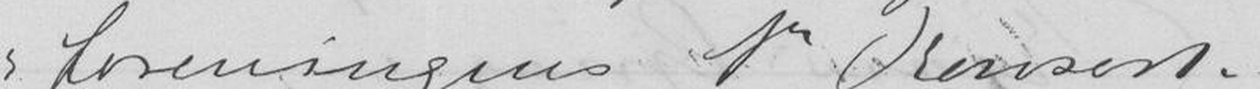foreningen 1te Konsert. -
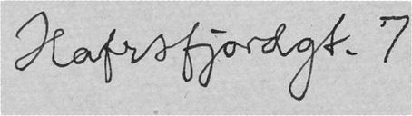Hafrsfjordgt. 7
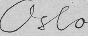Oslo
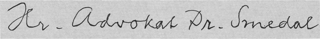Hr. Advokat Dr. Smedal
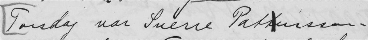Torsdag var Sverre Patursson
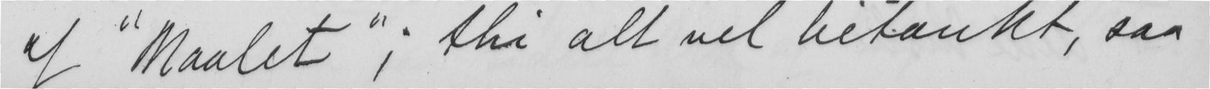af "Maalet"; thi alt vel betænkt, saa
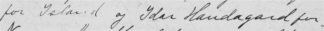for Island og Idar Handagard for
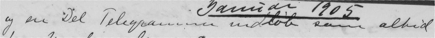og en Del Telegrammer udløb som altid
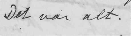Det var alt. x
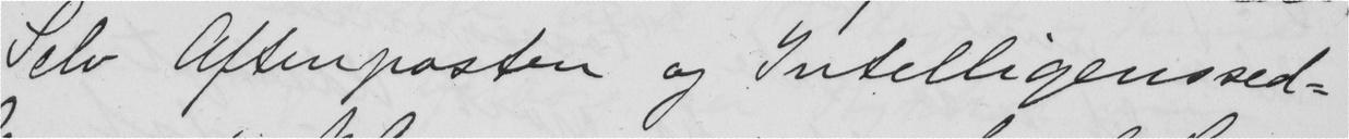Selv Aftenposten og Intelligenssed-
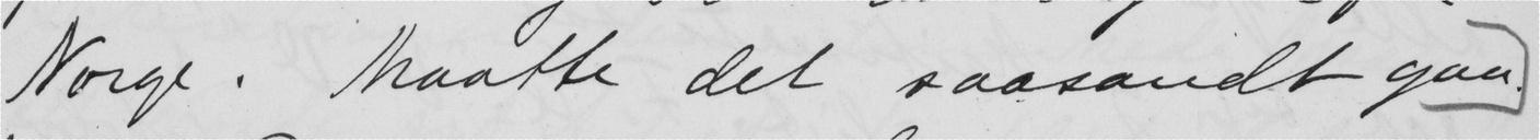Norge. Maatte det saasandt gaa
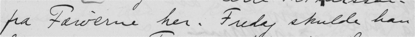fra Færøerne her. Fredag skulde han
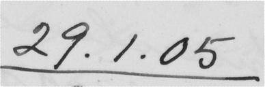29.1.05
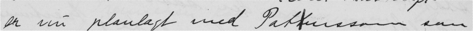er nu planlagt med Patursson som
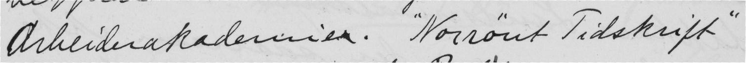Arbeiderakademier. "Norrønt Tidskrift"
